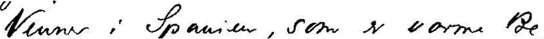Venner i Spanien, som er varme Be
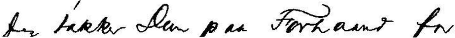Jeg takker Dem paa Forhaand for
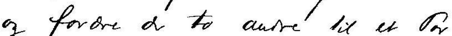og forære de to andre til et Par
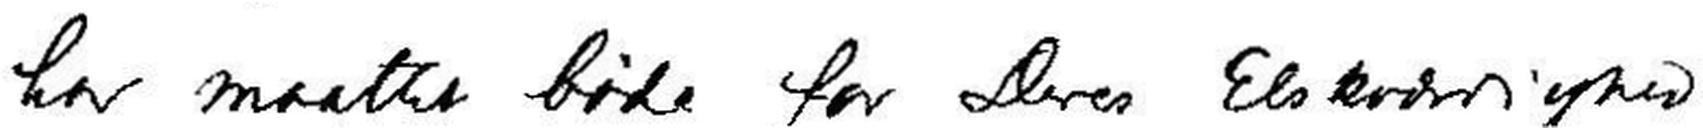har maattet bøde for Deres Elskværdighed
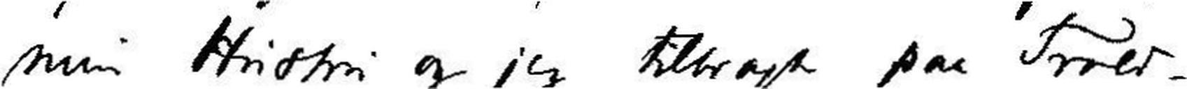min Hustru og jeg tilbragte paa Trold-
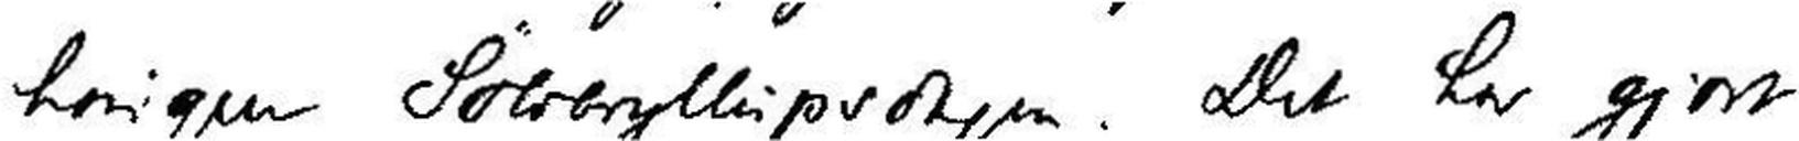haugen Sølvbryllupsdagen. Det har gjort
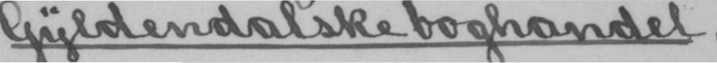Gyldendalske boghandel,
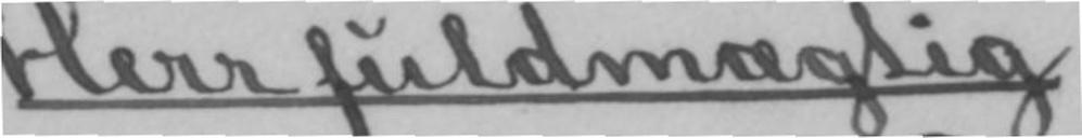Herr fuldmægtig
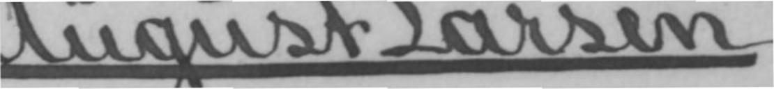August Larsen,
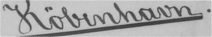København.
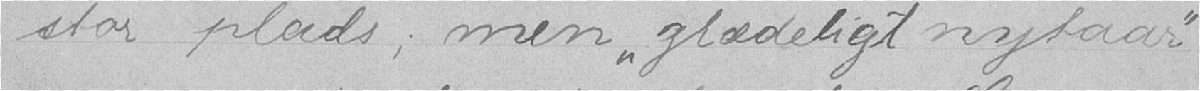stor plads; men "glædeligt nytaar"
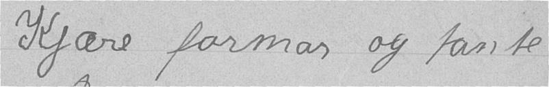Kjære farmor og tante
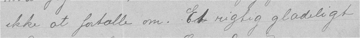ikke at fortælle om. Et rigtig glædeligt
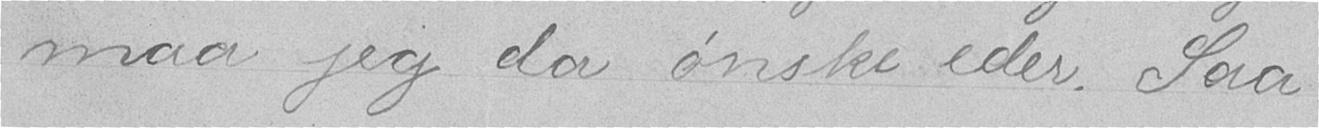maa jeg da ønske eder. Saa
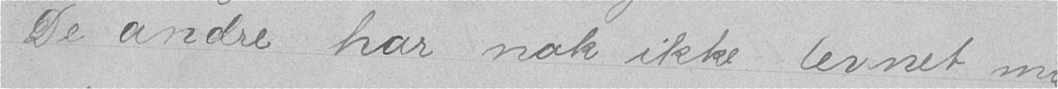De andre har nok ikke levnet mig
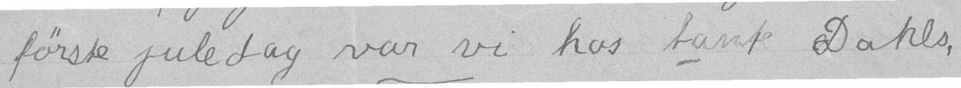første juledag var vi hos tante Dahls,
